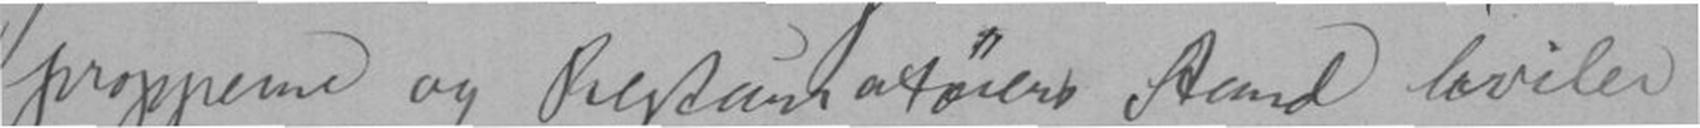propperne og Restauratørens Aand hviler
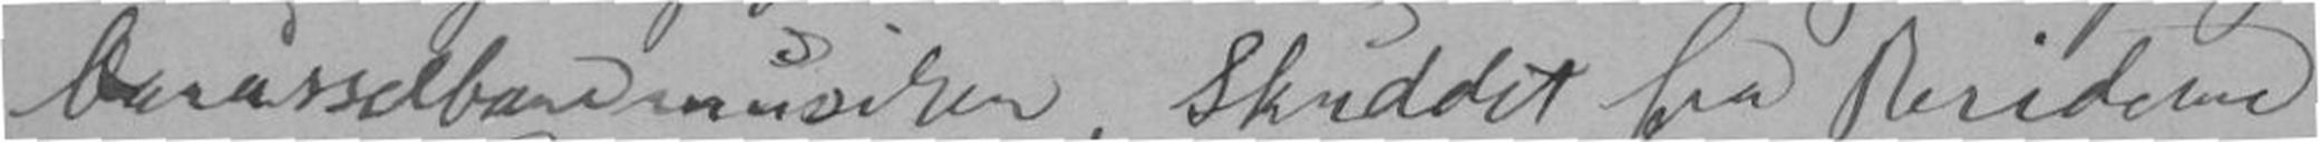Carusselbanemusiken, Skuddet fra Beriderne
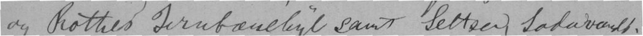og Rothes Jernbanehyl samt Seltser, Sodavands-
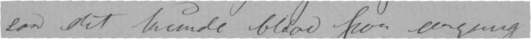som det kunde blive for engang
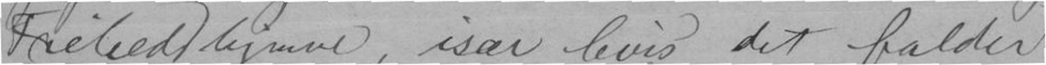Frihedshymne, især hvis det falder
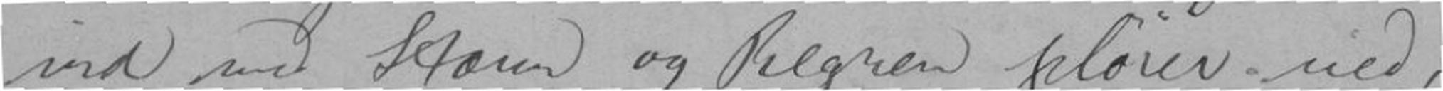ind med Storm og Regner pløier ned,
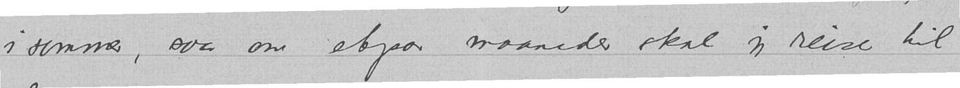i sommer, saa om etpar maaneder skal jeg reise til
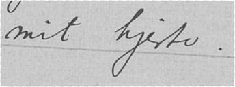mit hjerte.
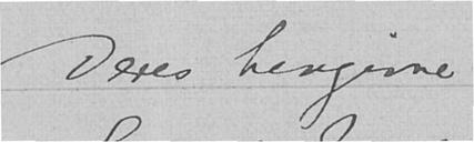Deres hengivne
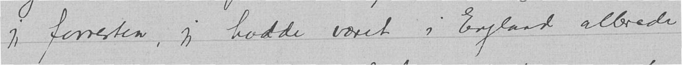jeg forresten, jeg hadde været i England allerede
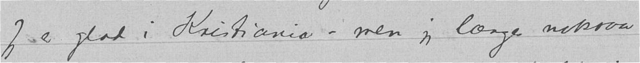Jeg er glad i Kristiania - men jeg længes noksaa
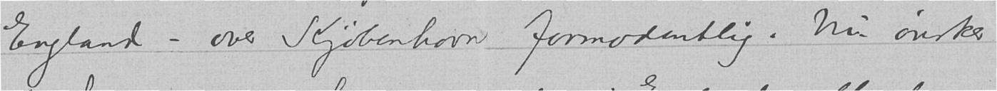England – over Kjøbenhavn formodentlig. Nu ønsker
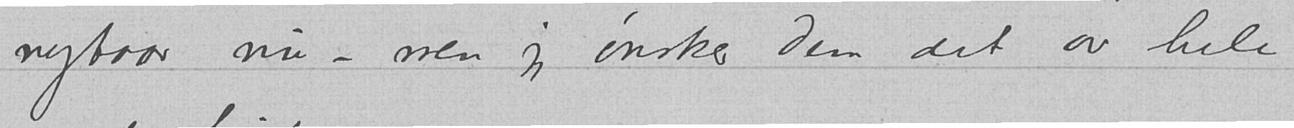nytaar nu – men jeg ønsker Dem det av hele
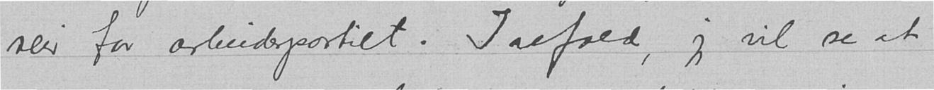seir for arbeiderpartiet. Ialfald, jeg vil se at
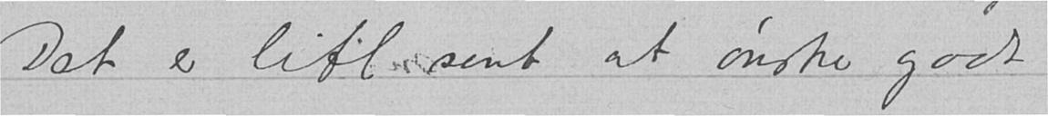Det er litt sent at ønske godt
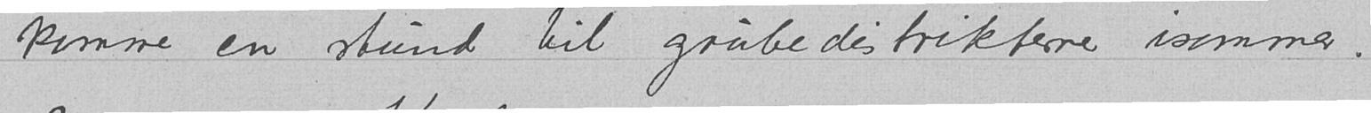komme en stund til grubedistrikterne isommer.
